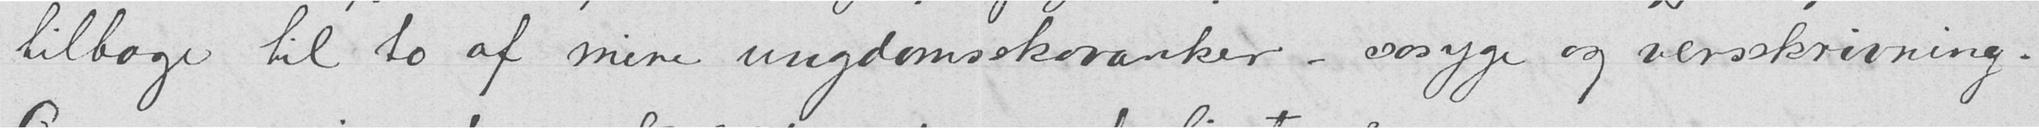tilbage til ro af mine ungdomsskavanker - søsyge og versskrivning.
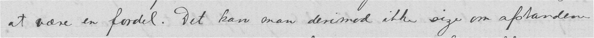at være en fordel. Det kan man derimod ikke sige om afstanden
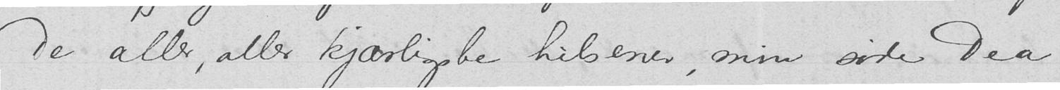De aller, aller kjærligste hilsener, min søde Dea
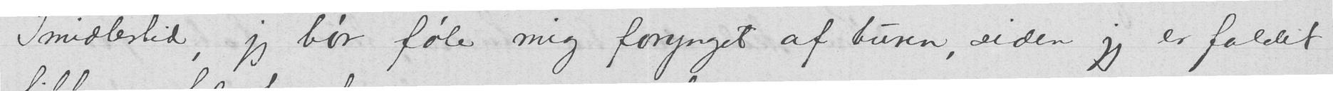Imidlertid, jeg bør føle mig forynget af turen, siden jeg er faldet
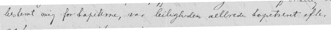bestemt meg for tapeterne, var leiligheden allerede tapetseret efter
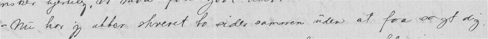Nu har jeg atter skrevet to sider sammen uden at faa sagt dig,
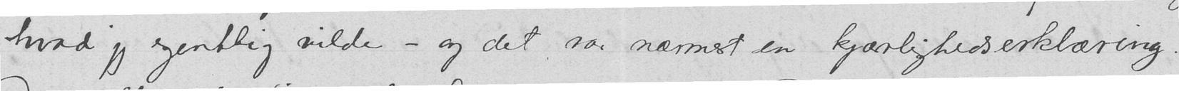hvad jeg egentlig vilde - og det var nærmest en kjærlighedserklæring.
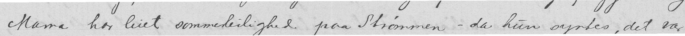Mama har leiet sommereilighed paa Strømmen - da hun syntes, det var
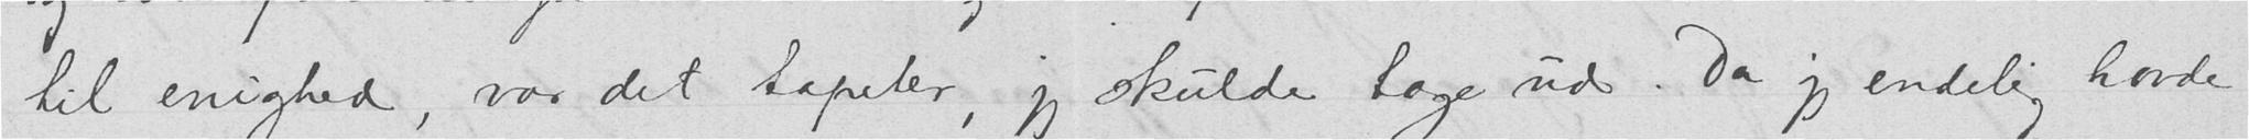til enighed, var det tapeter, jeg skulde tage ud. Da jeg endelig havde
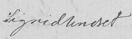Sigrid Undset
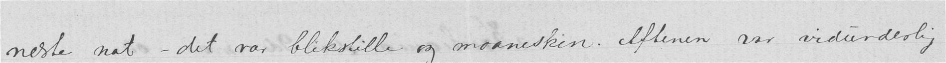neste nat - det var blikstille og maaneskin. Aftenen var vidunderlig
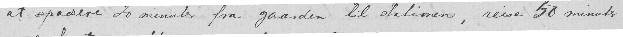at spadsere 20 minutter fra gaarden til stationen, reise 50 minutter
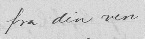fra din ven
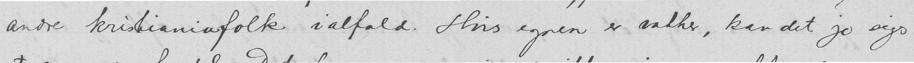andre kristianiafolk ialfald. Hvis egnen er vakker, kan det jo siges
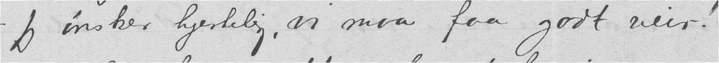Jeg ønsker hjertelig, vi maa faa godt veir!
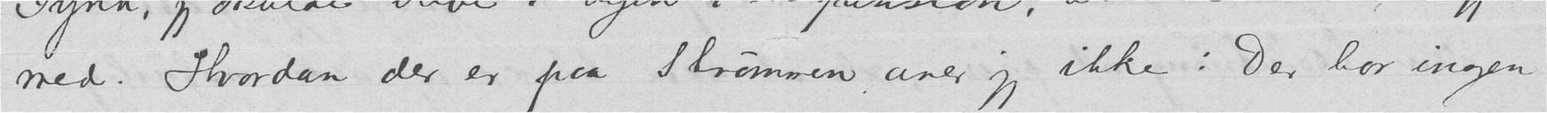med. Hvordan der er paa Strømmen aner jeg ikke: Der bor ingen
